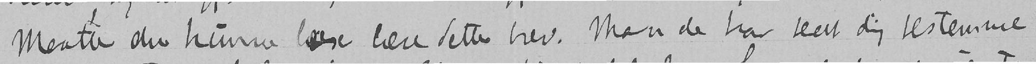Maatte du kunne læse læse dette brev. Mon de har bedt dig bestemme
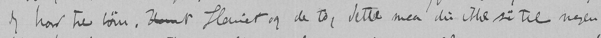Jeg har tre børn, Harret Harriet og de to, dette maa du ikke si til nogen.
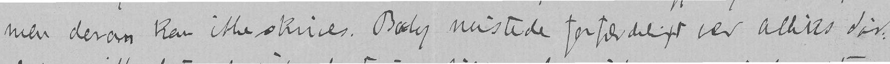men derom kan ikke skrives. Baby mistede forfærdeligt ved Alliks død.
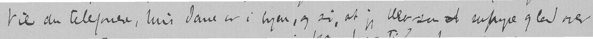Vil du telefonere, hvis Jane er i byen, og si, at jeg blev saa et uhyre glad over
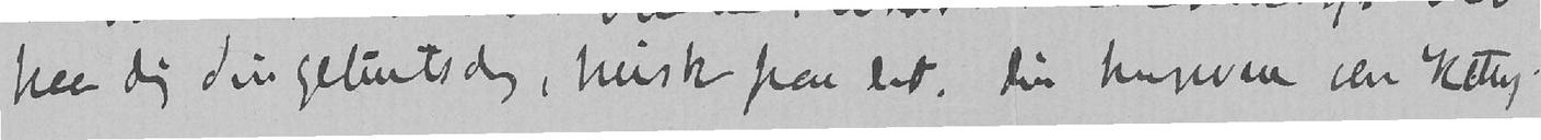paa dig din geburtsdag, husk paa det. Din hengivne ven Kitty.
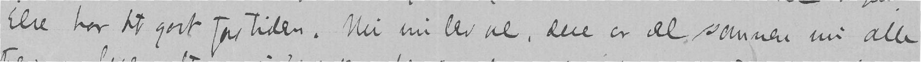Else har det godt for tiden. Nei nu lev vel, dere er vel sammen nu alle
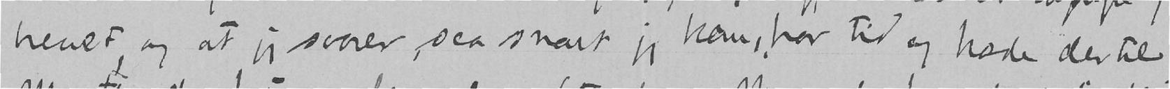brevet, og at jeg svarer, saa snart jeg kan, har tid og hode dertil.
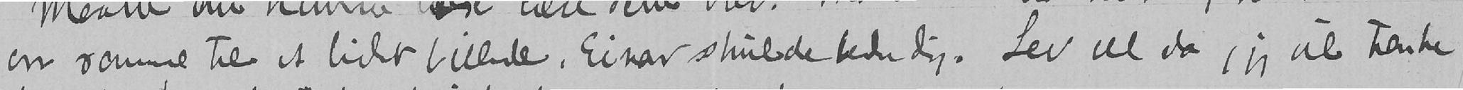en ramme til et lidet billede, Einar skulde bede dig. Lev vel da, jeg vil tænke
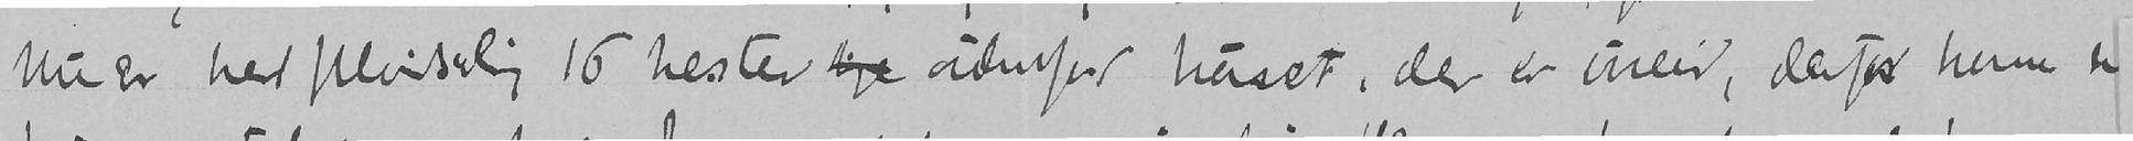Nu er her pludselig 16 hester lige udenfor huset, der er uveir, derfor kom de.
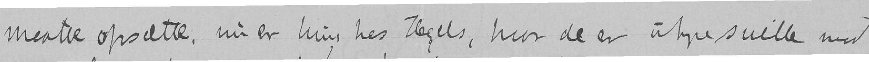maatte opsætte, nu er hun hos Hegels, hvor de er uhyre snille mod
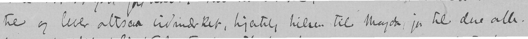tre og lever altsaa udmærket, hjertelig hilsen til Magda, ja til dere alle.
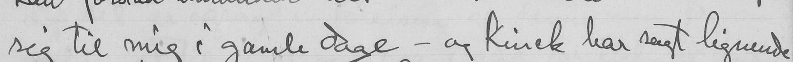sig til mig i gamle dage - og Kinck har sagt lignende
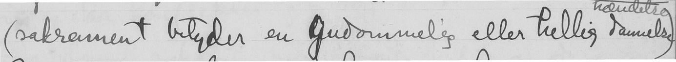(sakrement betyder en gudommelg eller hellig dannelse)
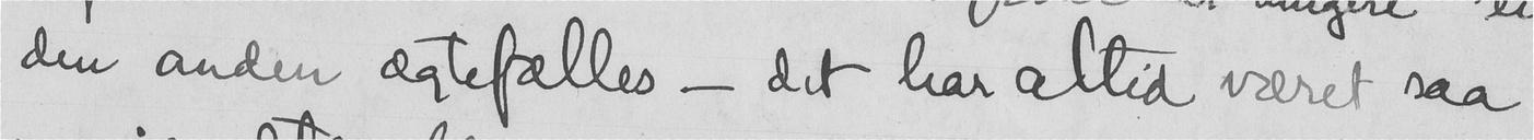den anden ægtefælles - det har altid været saa
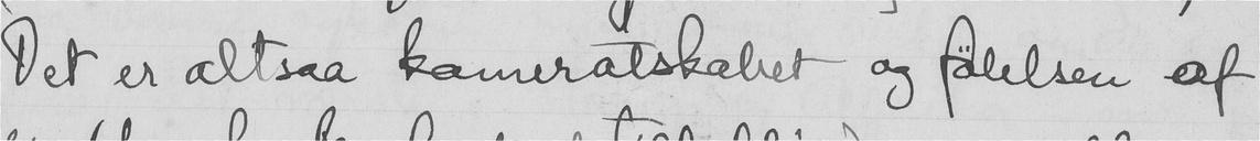Det er altsaa kameratskabet og følelsen af
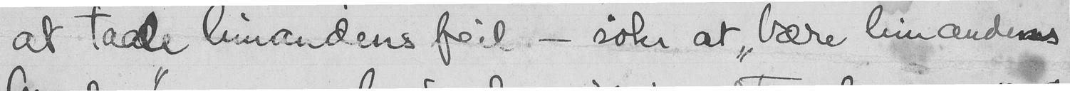at taale hinandens feil, - søke at "bære hinandens
